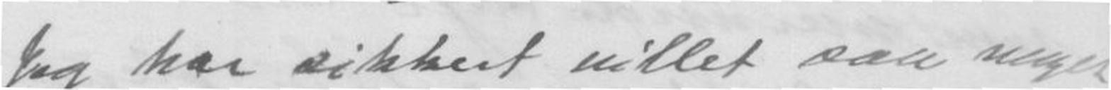Jeg har sikkert villet saa meget,
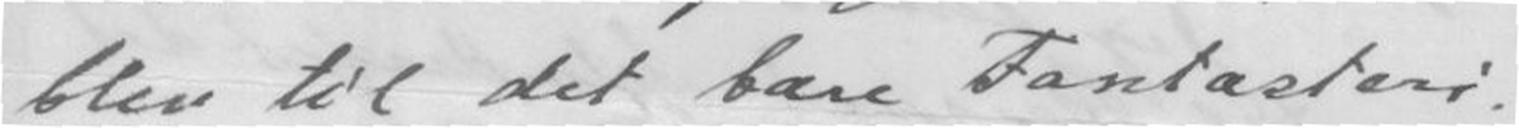blev til det bare Fantasteri.
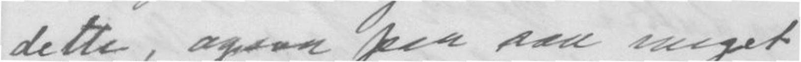dette, ogsaa paa saa meget
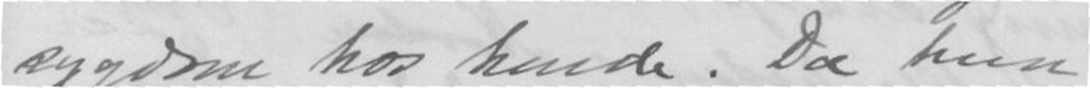sygdom hos hende. Da hun
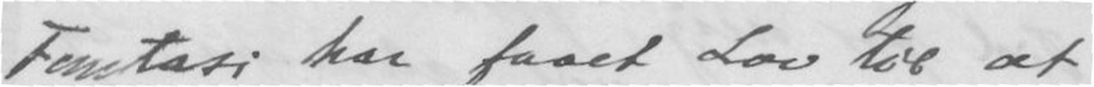Fantasi har faaet Lov til at
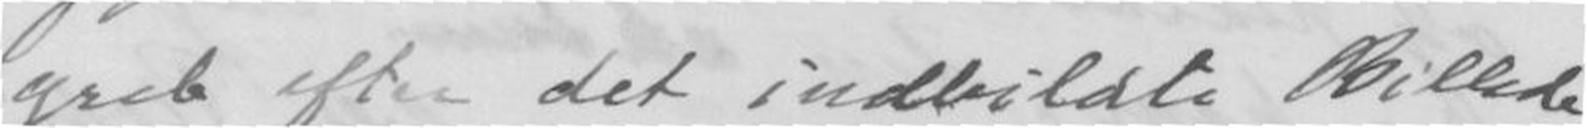greb efter det indbildte Billede
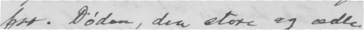for. Døden, den store og ædle,
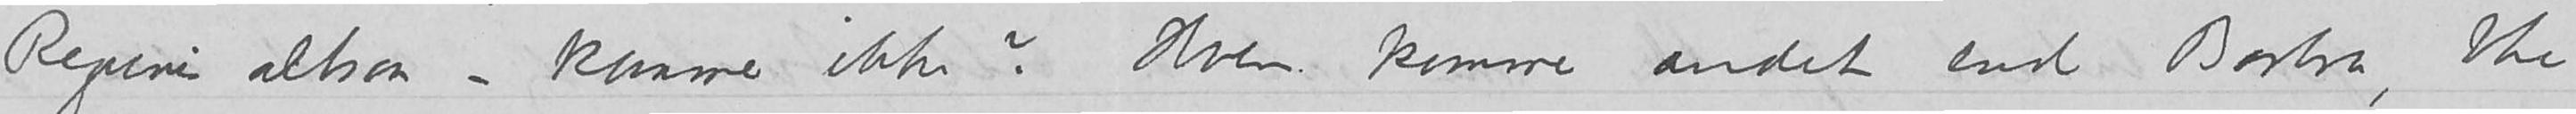hendes – Regines altsaa –- kommer ikke?  Hvem kommer andet end Barbra, the
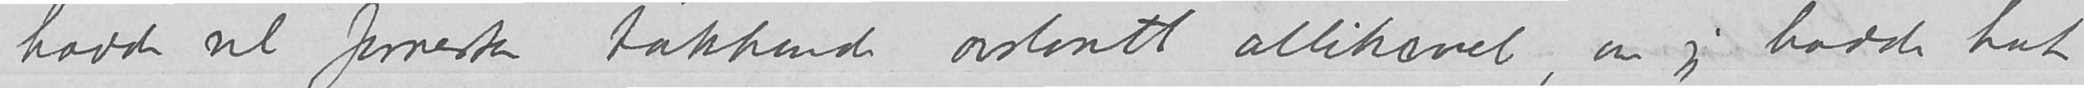Jeg hadde vel forresten takkende avslaatt allikæevel, om jeg hadde hat
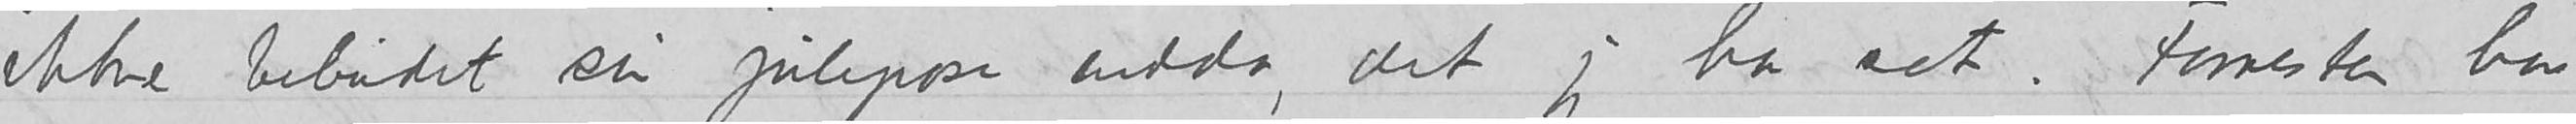ikke bebudet sin julepose endda, det jeg har set. Forresten har
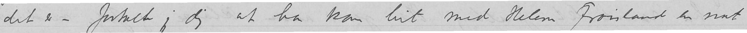det er – fortalte jeg dig at han kom hit med Helene Frøisland en nat
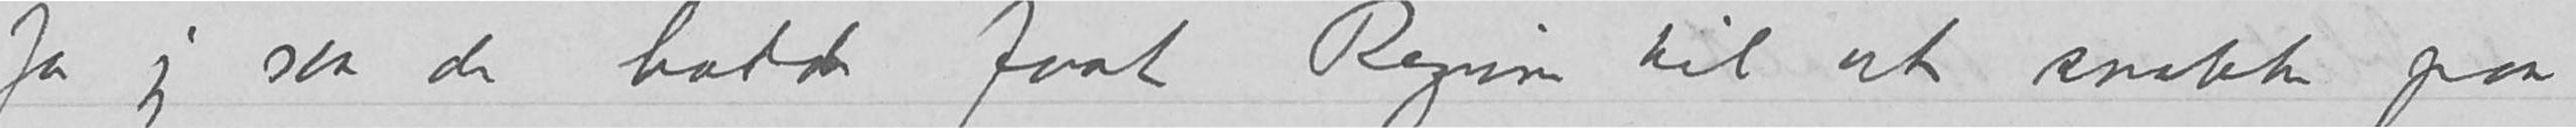Ja jeg saa de hadde faat Regine til at snakke paa
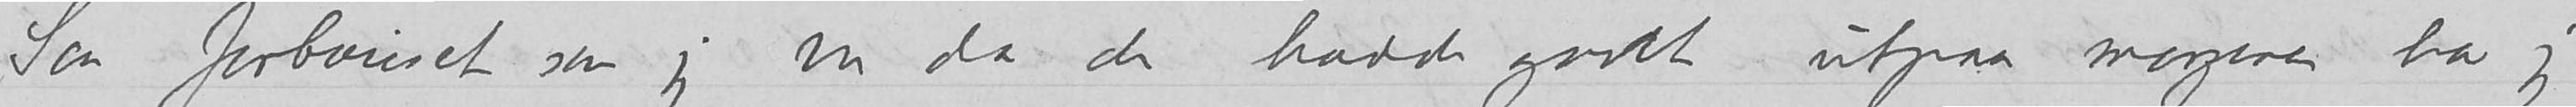Saa forbauset som jeg var da de hadde gaat utpaa morgenen har jeg
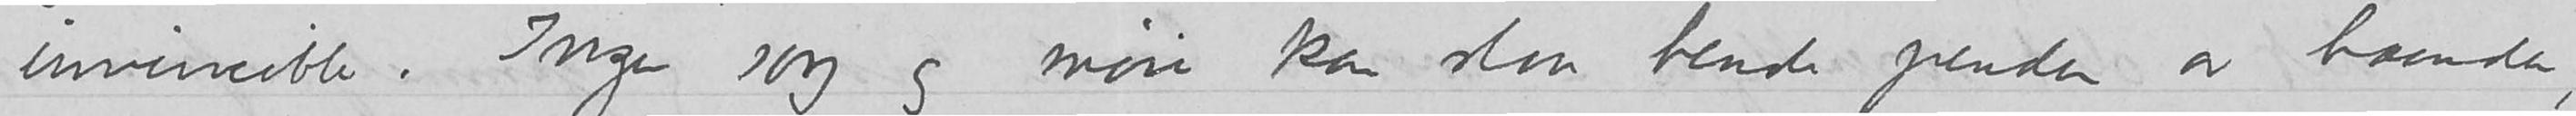invincible. Ingen sorg og møie kan slaa hende penden av haanden,
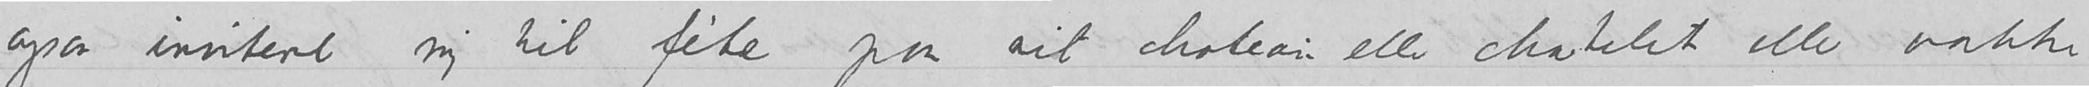ogsaa inviteret mig til fèéte paa sit chateau eller chatelet eller vakke
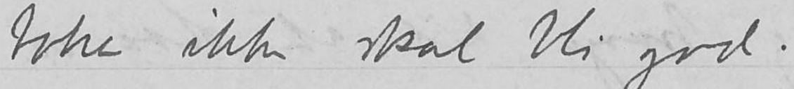boken ikke skal bli god.
